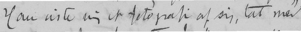Han viste mig et fotografi af sig, tat med
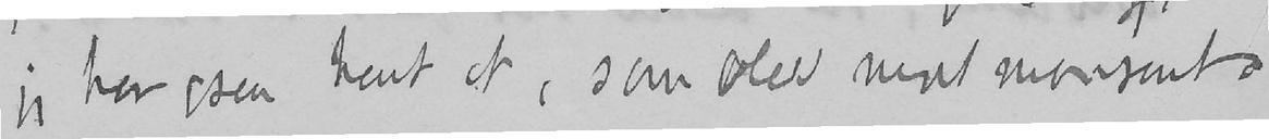jeg har ogsaa havt et, som blev meget morsomt.
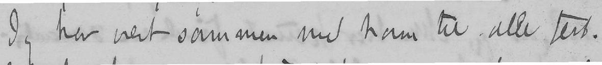Jeg har vært sammen med ham til alle fest-
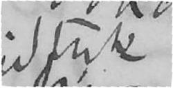udtryk
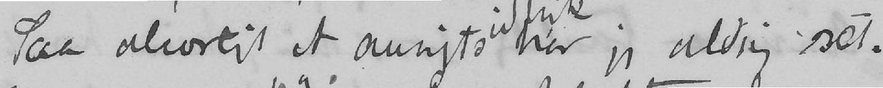Saa alvorligt et ansigts har jeg aldrig set.
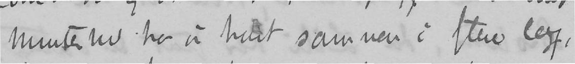og munterhed har vi havt sammen i flere lag,
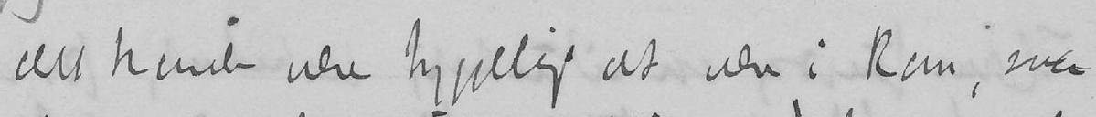det kunde være hyggeligt at være i Rom, saa
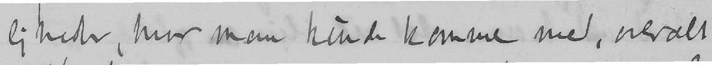ligheder, hvor man kunde komme med, overalt
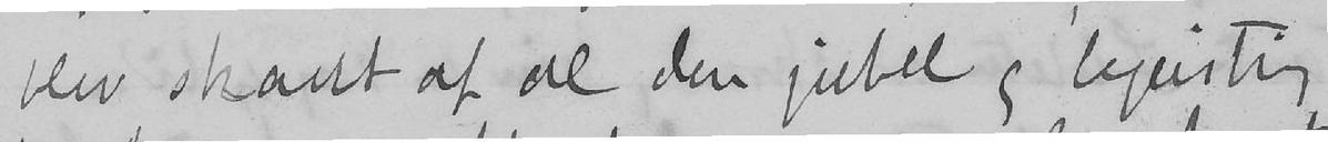blev skadet af al den jubel og begeistring
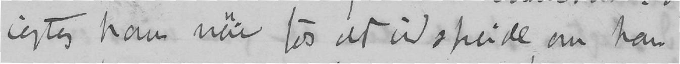iagtog ham nøie for at udspeide, om han
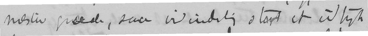næsten græde, saa vidunderlig stort et udtryk
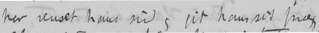har renset hans sind og git ham sit præg.
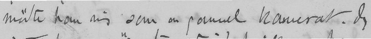mødte han mig som en gammel kamerat. Jeg
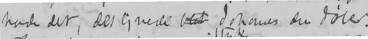havde det, det lignede blot Johanes den døber.
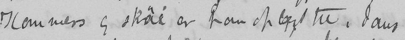Kommers og skøi er han oplært til, dans
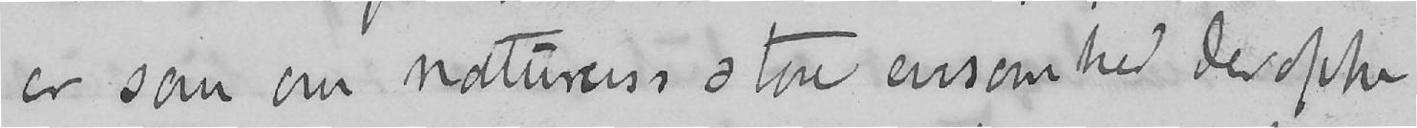er som om naturens store ensomhed deroppe
